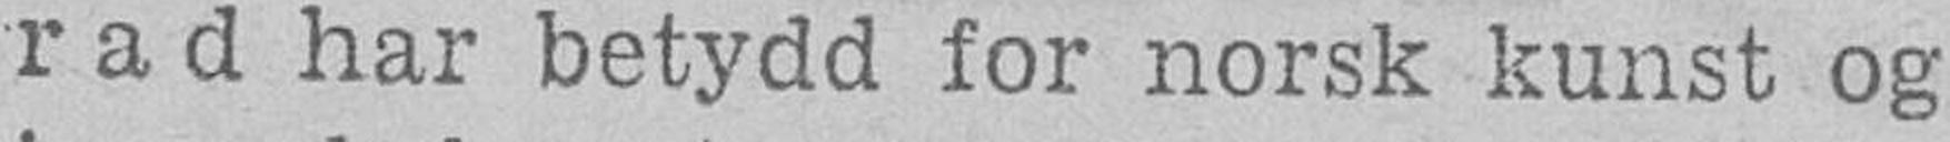rad har betydd for norsk kunst og
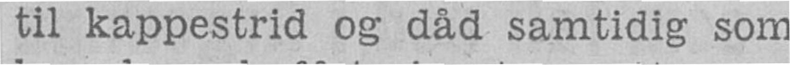til kappestrid og dåd samtidig som
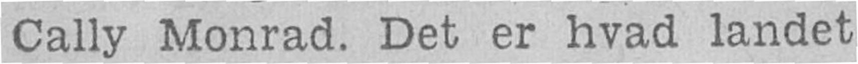Cally Monrad. Det er hvad landet
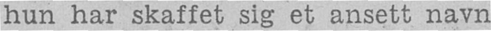hun har skaffet sig et ansett navn
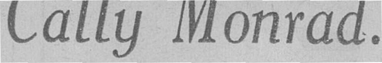Cally Monrad.
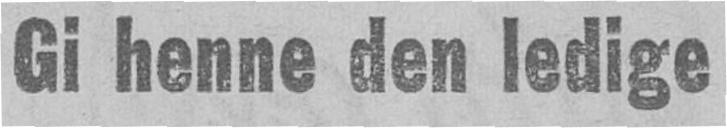Gi henne den ledige
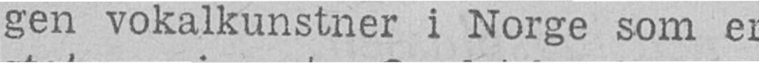gen vokalkunstner i Norge som er
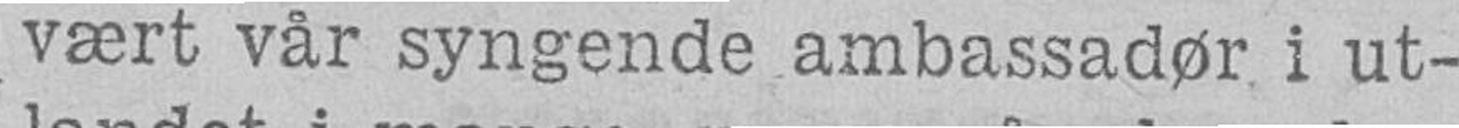vært vår syngende ambassadør i ut-
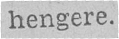hengere.
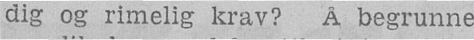dig og rimelig krav? Å begrunne
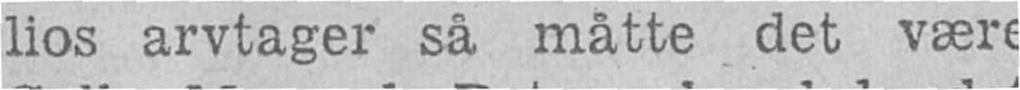lios arvtager så måtte det være
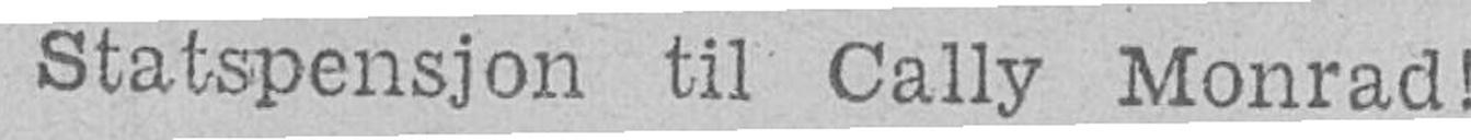Statspensjon til Cally Monrad!
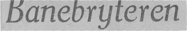Banebryteren
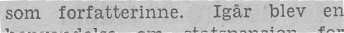som forfatterinne. Igår blev en
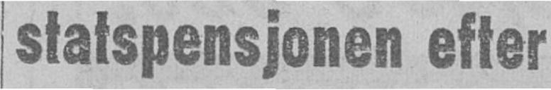statspensjonen efter
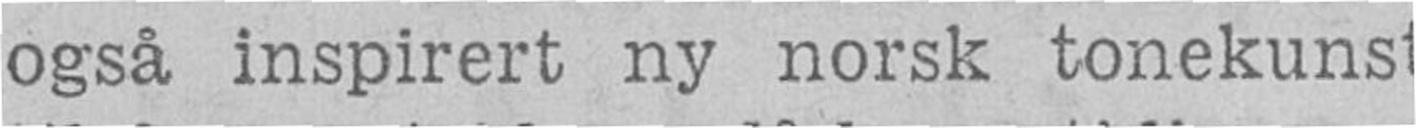også inspirert ny norsk tonekunst
